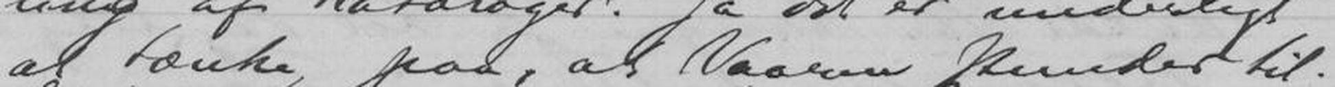at tænke paa, at Vaaren stunder til.
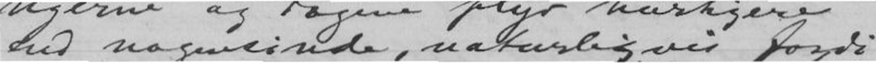end nogensinde, naturligvis fordi
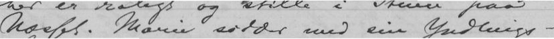Næsset. Marie sidder med sin Yndlings-
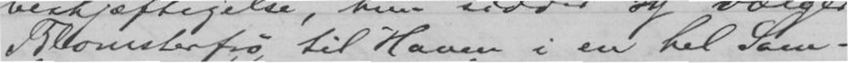Blomsterfrø til Haven i en hel Sam-
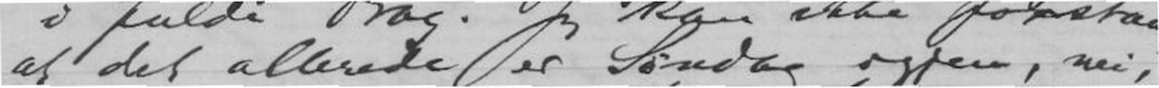at det allerede er Søndag igjen, nei,
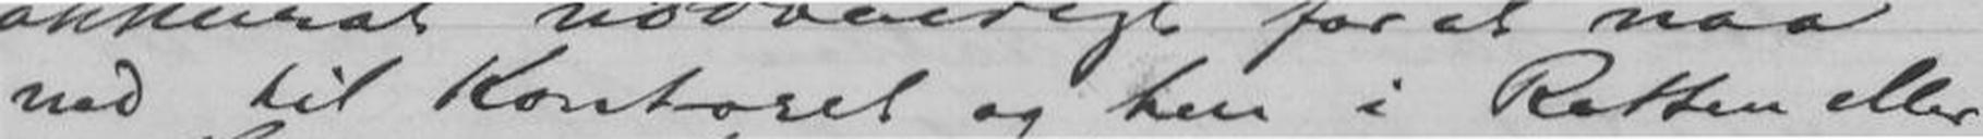ned til Kontoret og hen i Retten eller
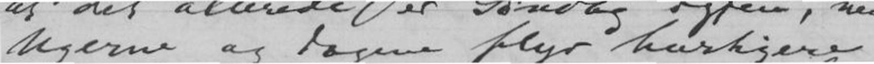Ugerne og Dagene flyr hurtigere
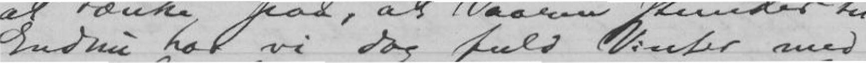Endnu har vi dog fuld Vinter med
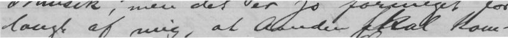langt af mig, at Aanden skal kom-
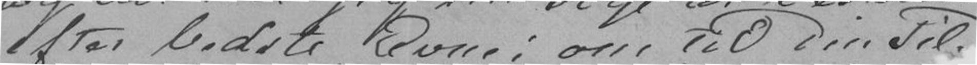efter bedste Evne; om Til Din Til-
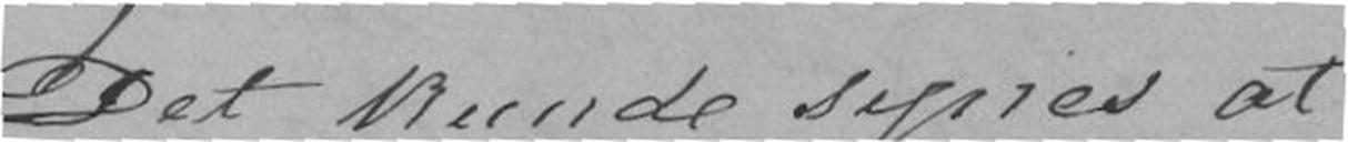Det kunde synes at
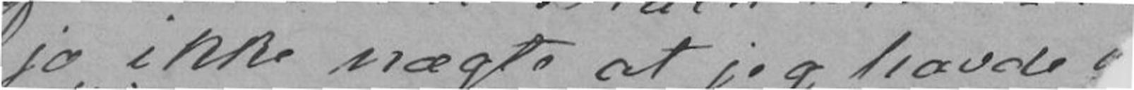jo ikke nægte at jeg havde
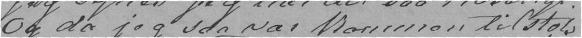Og da jeg saa var kommen tilstols
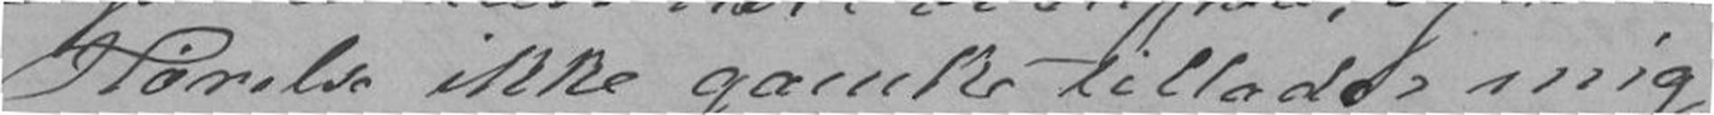Hørelse ikke ganske tillader mig
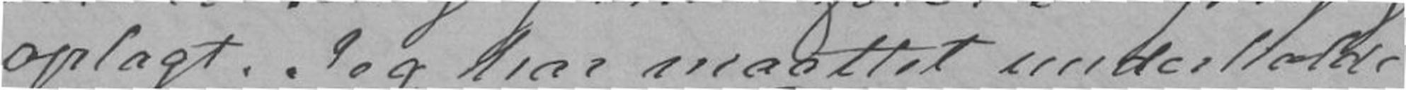oplagt. Jeg har maattet underholde
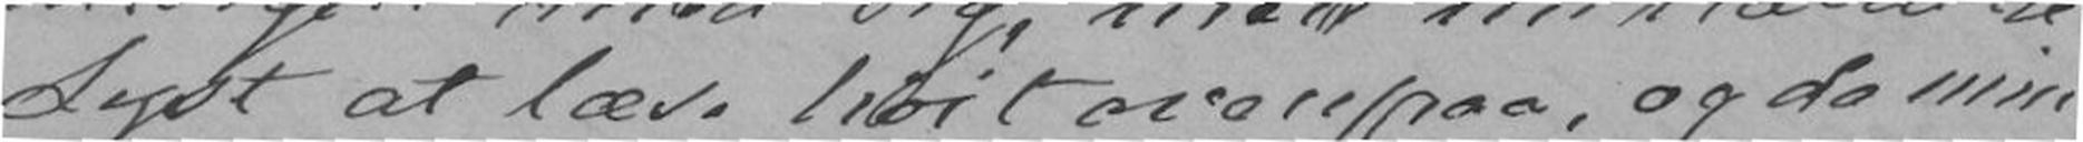Lyst at læse høit ovenpaa, og da min
